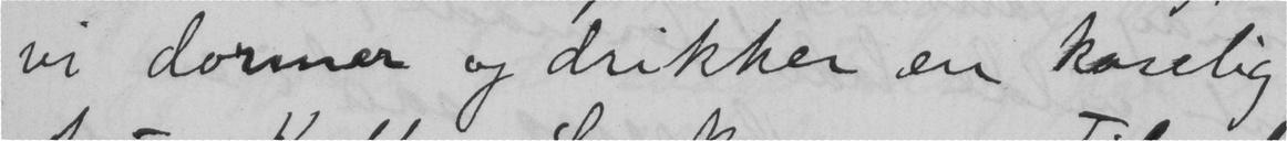vi dormer og drikker en koselig
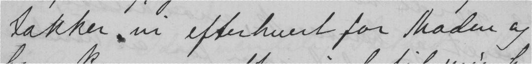takker vi efterhvert for Maden og
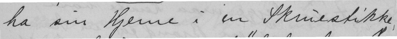ha sin Hjerne i en Skruestikke,
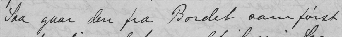Saa gaar den fra Bordet som først
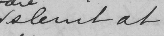slemt at
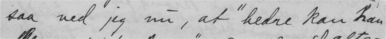saa ved jeg nu, at "bedre kan kan
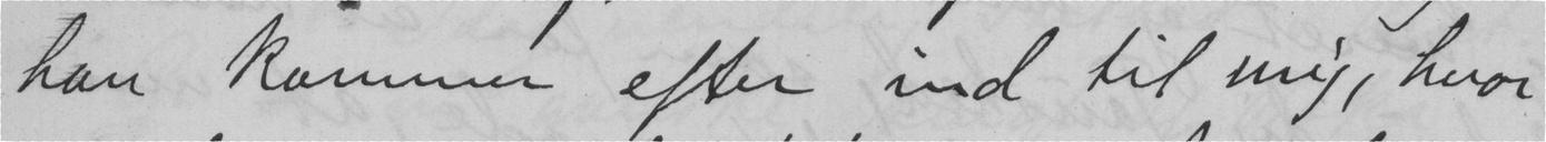han kommer efter ind til mig, hvor
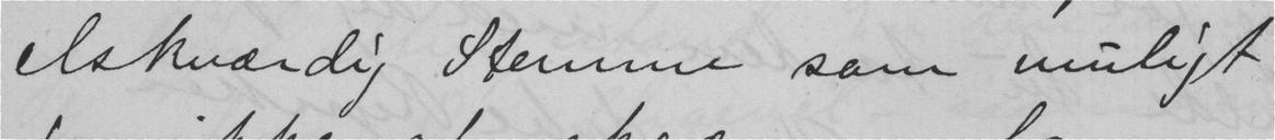elskværdig Stemme som muligt
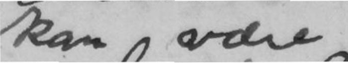kan være
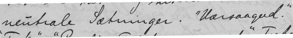neutrale Sætninger. "Værsaagod."
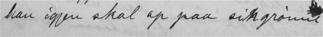han igjen skal op paa sit grønne
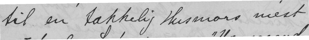til en tækkelig Husmors mest
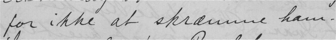for ikke at skræmme ham.
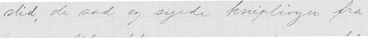slid, de sad og syede kniplinger fra
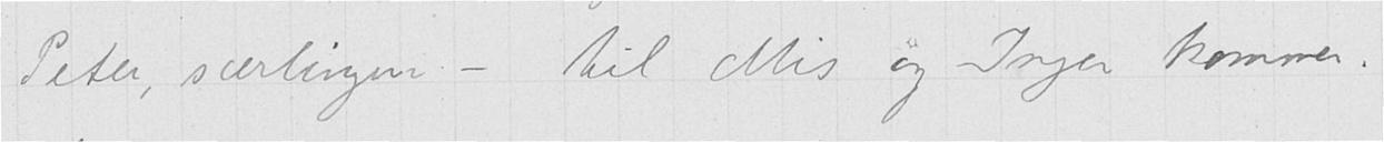Peter, særlingen - til Mis og Inger kommer.
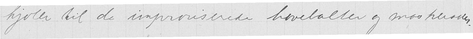kjoler til de improviserede haveballer og maskerader.
